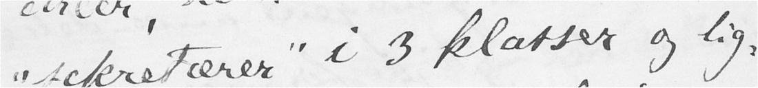"sekretærer" i 3 klasser og lig-
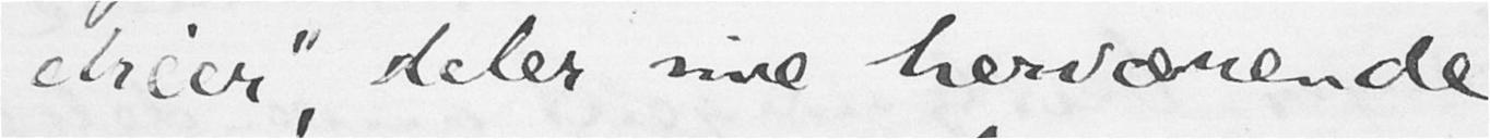chéer", deler sine herværende
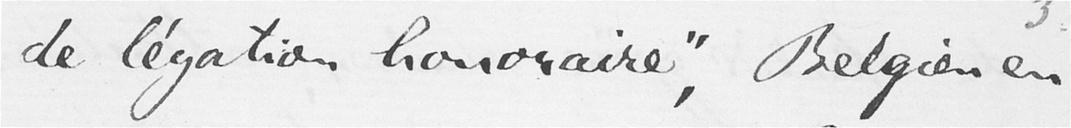de légation honoraire", Belgien en
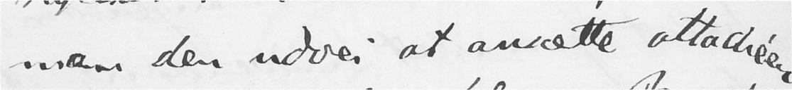man den udvei at ansætte attachéer
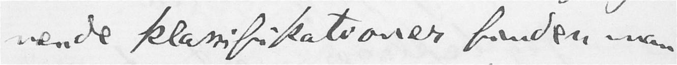nende klassifikationer finder man
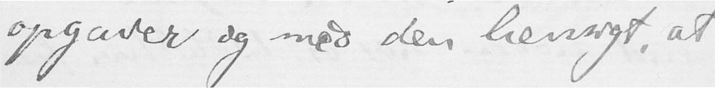opgaver og med den hensigt, at
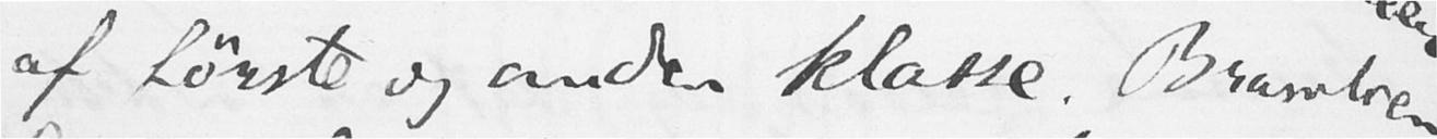af første og anden klasse. Brasilien
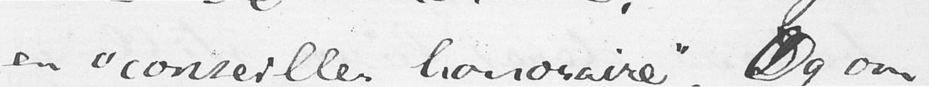en "conseiller honoraire". Og om
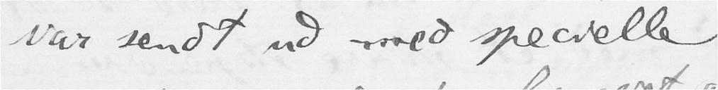var sendt ud med specielle
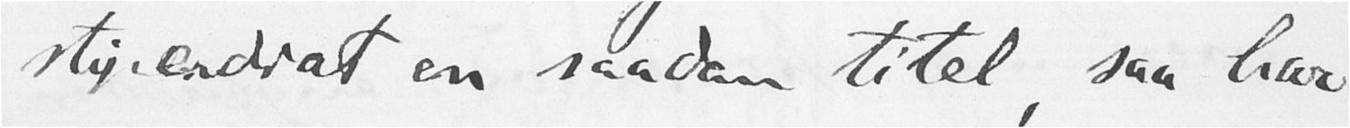stipendiat en saadan titel, saa har
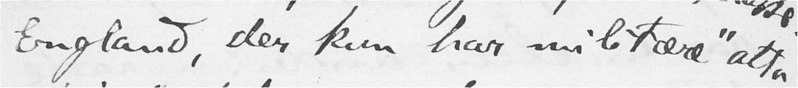England, der kun har militære "atta-
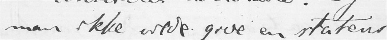man ikke vilde give en statens
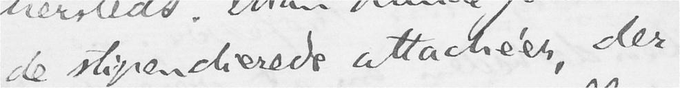de stipendierede attachéer, der
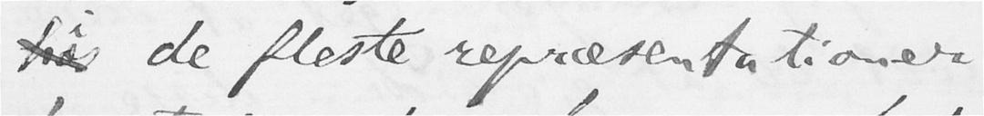i de fleste repræsentationer
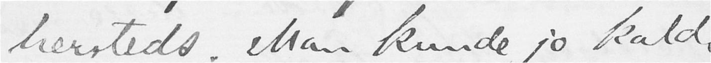hersteds. Man kunde jo kalde
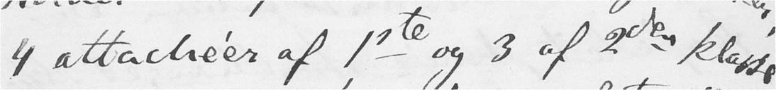4 attachéer af 1ste og 3 af 2den klasse.
